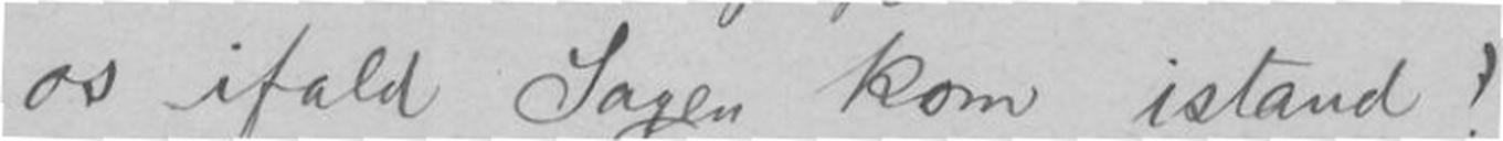os ifald Sagen kom istand!
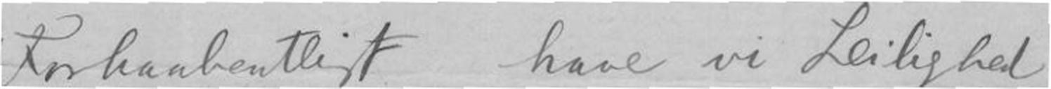Forhaabentligt have vi Leilighed
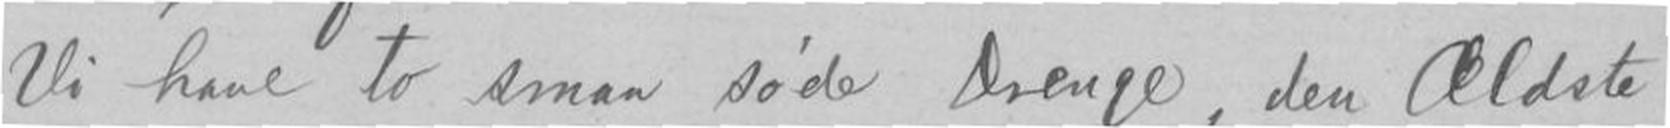Vi have to smaa søde Drenge, den Ældste
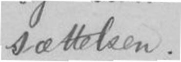settelsen.
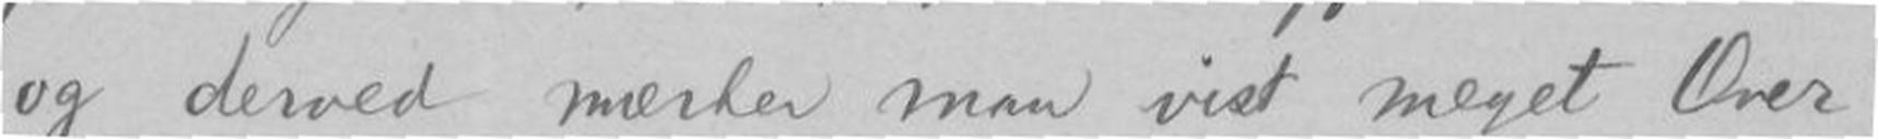og derved mærker man vist meget Over
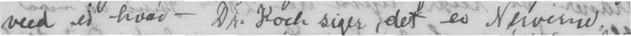veed ei hvad - Dr. Koch siger, det er Nerverne.
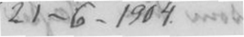21 - 6 - 1904.
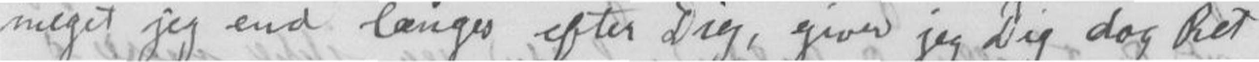meget jeg end længes efter Dig, giver jeg Dig dog Ret
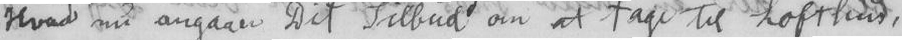Hvad nu angaaer Dit Tilbud om at tage til Lofthus,
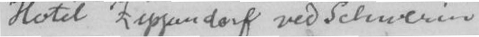Hotel Zippendorf ved Schwerin
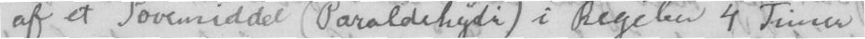af et Sovemiddel Paraldehydi) i Regelen 4 Timer
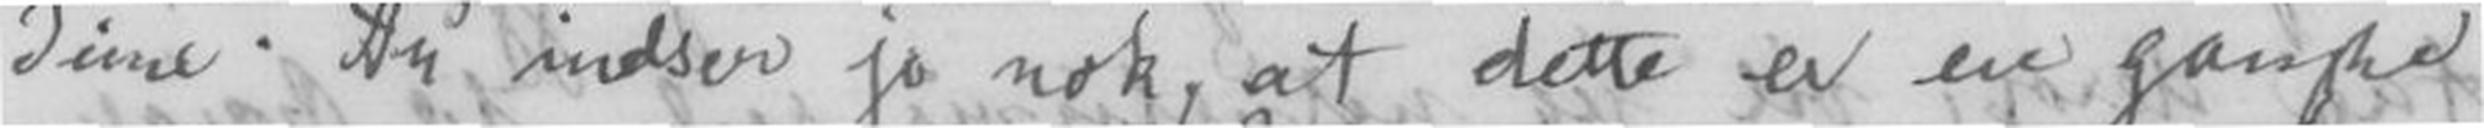Time. Du indser jo nok, at dette er en ganske
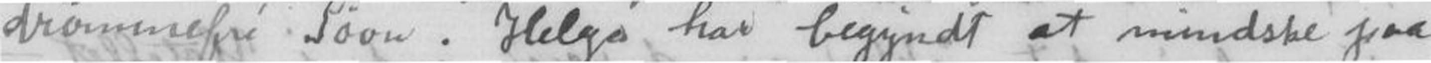drømmefri Søvn. Helga har begyndt at mindske paa
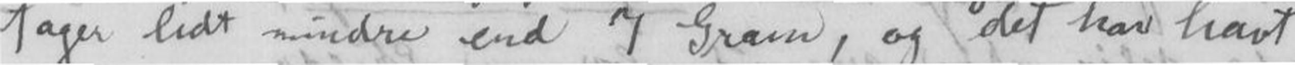tager lidt mindre end 7 Gram, og det har havt
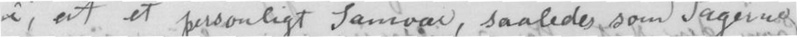i, at et personligt Samvær, saaledes som Sagerne
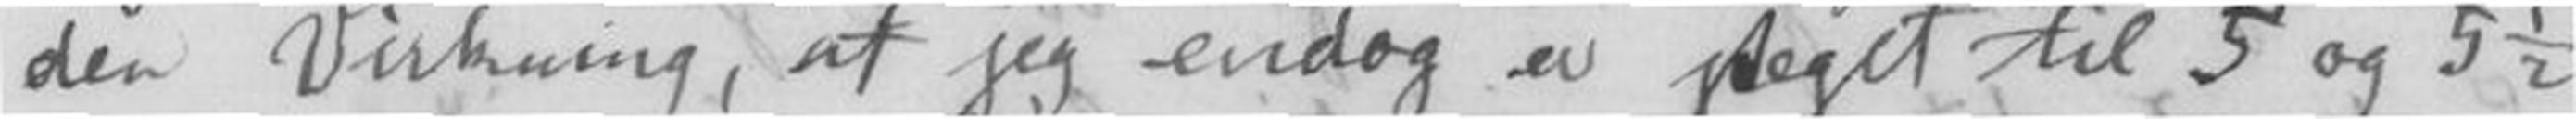den Virkning, at jeg endog er steget til 5 og 5 1/2
